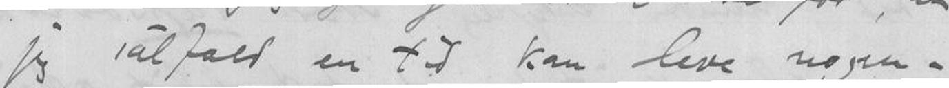jeg ialfald en tid kan leve nogen-
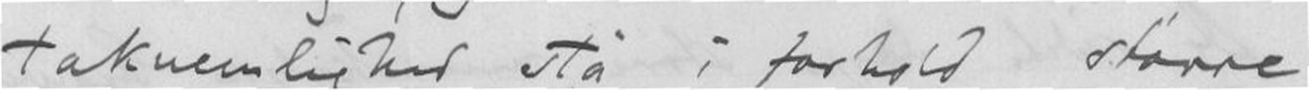taknemlighed stå i forhold, større
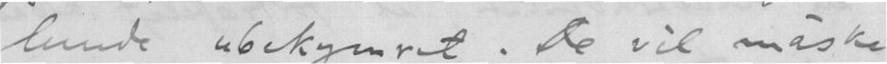lunde ubekymret. De vil måske
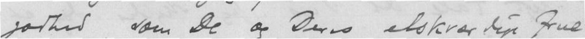godhed, som De og Deres elskværdige frue
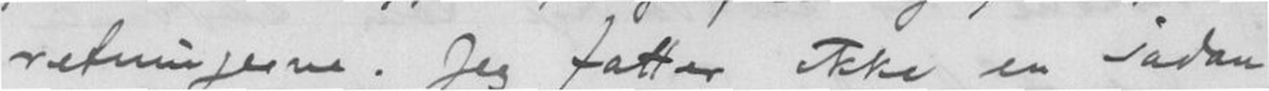retningen. Jeg fatter ikke en sådan
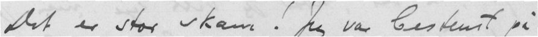Det er stor skam! Jeg var bestemt på
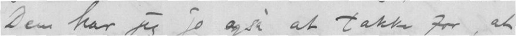Dem har jeg jo også at takke for, at
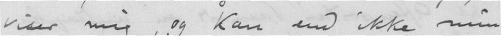viser mig, og kan end ikke min
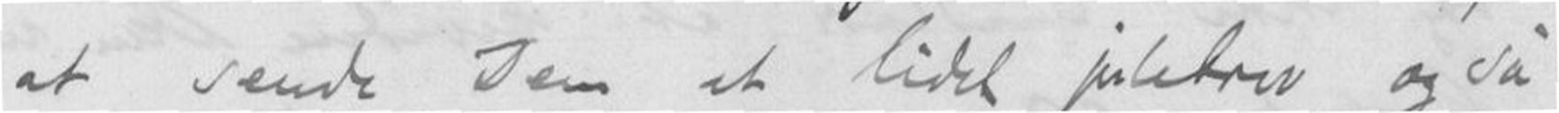at sende Dem et lidet julebrev og så,
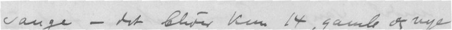med sange - det bliver kun 14 gamle og nye
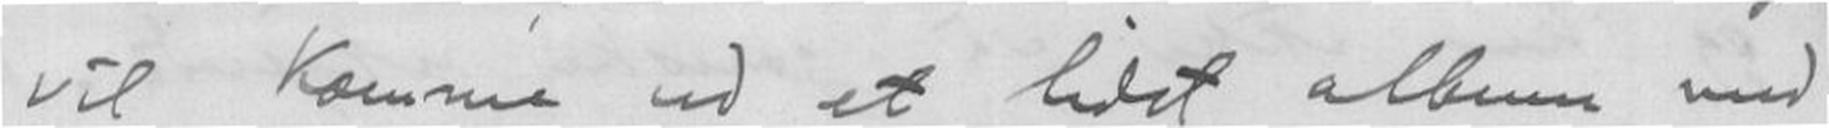vil komme ud et lidet album
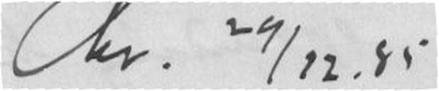Chr. 29/12.85
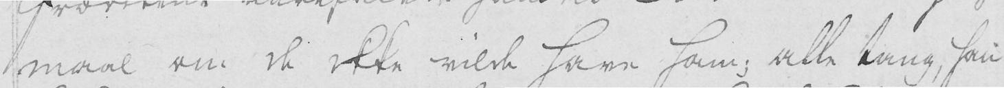maal om de ikke vilde have ham; alle taug, han
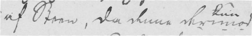af Steen, da denne derimod
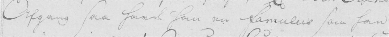Afgang saa havde han en famulus som han
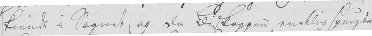kiendt i Sognet, og da Biskoppen endelig spurgte
